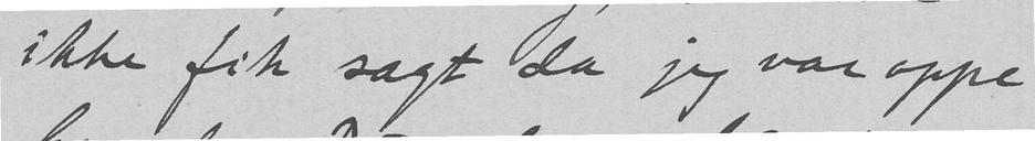ikke fik sagt da jeg var oppe
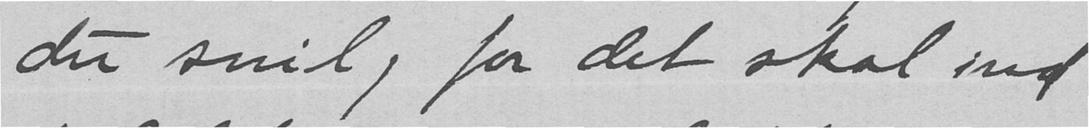du snil, for det skal ind
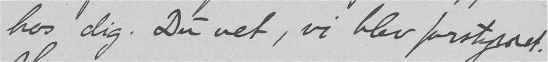hos dig. Du vet, vi blev forstyrret.
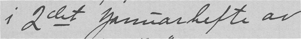i 2det Januarhefte av
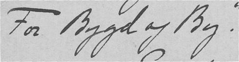For Bygt og By.
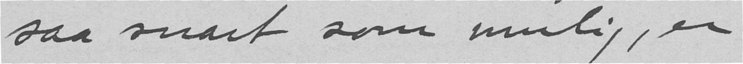saa snart som mulig, er
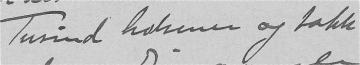Tusind hilsner og takk
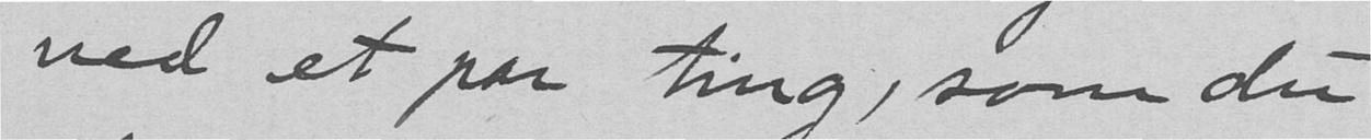ned et par Ting, som du
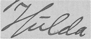Hulda
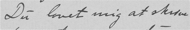Du lovet mig at skrive
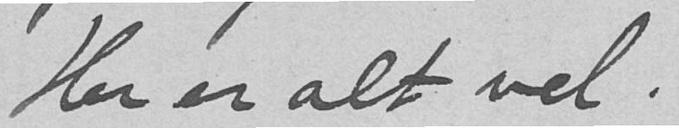Her er alt vel.
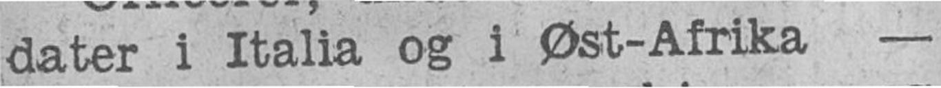dater i Italia og i Øst-Afrika -
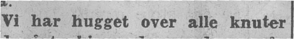Vi har hugget over alle knuter
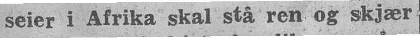seier i Afrika skal stå ren og skjær
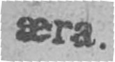æra.
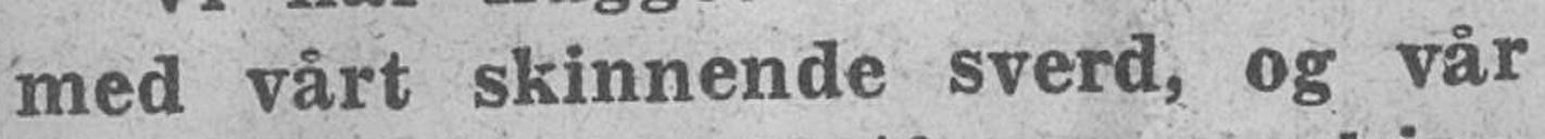med vårt skinnende sverd, og vår
